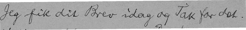Jeg fik dit Brev idag og Tak for det.
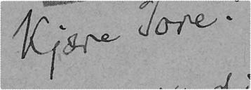Kjære Tore!
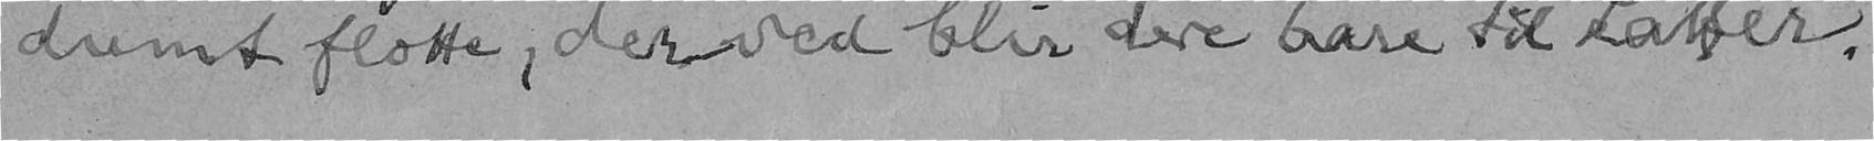dumt flotte, derved blir dere bare til Latter.
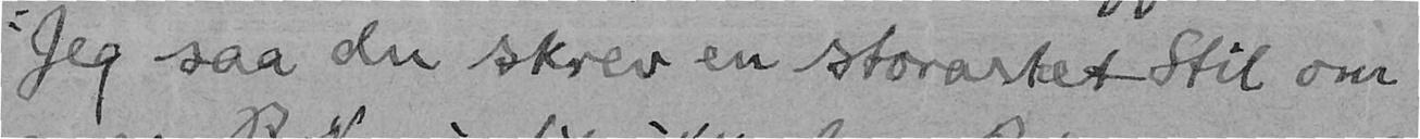Jeg saa du skrev en storartet Stil om
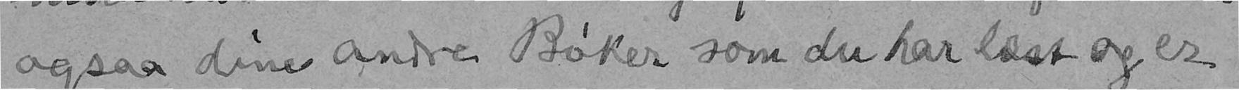ogsaa dine andre Bøker som du har læst og er
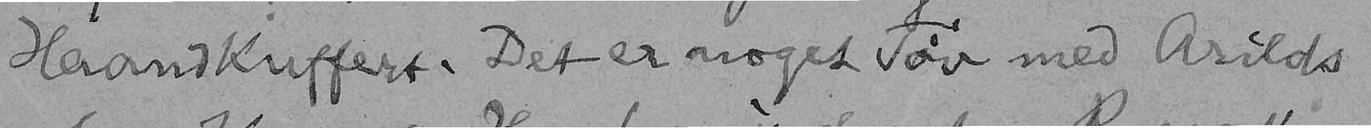Haandkuffert. Det er noget Tøv med Arilds
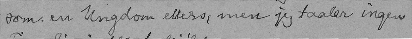som en Ungdom ellers, men jeg taaler ingen
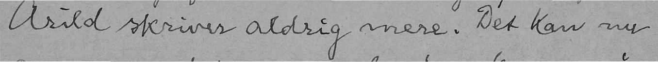Arild skriver aldrig mere. Det kan nu
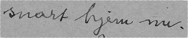snart hjem nu.
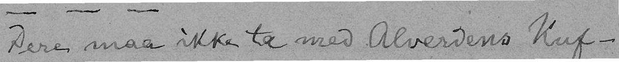Dere maa ikke ta med Alverdens Kuf-
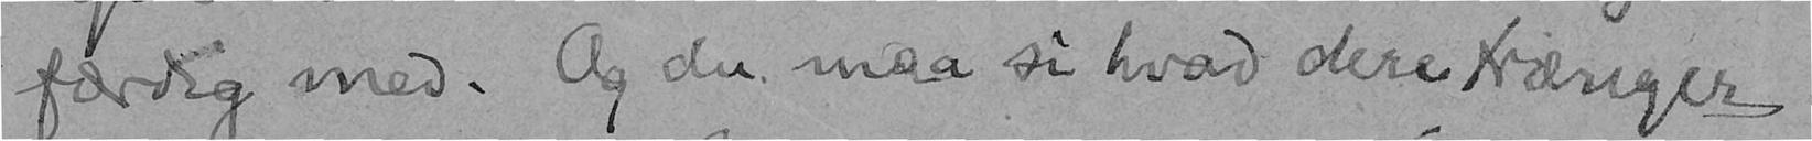færdig med. Og du maa si hvad dere trænger
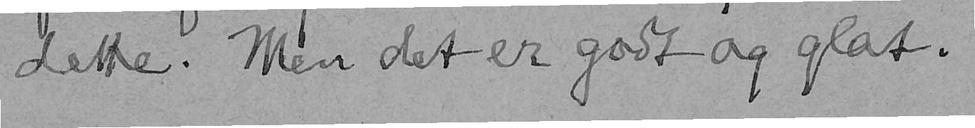dette. Men det er godt og glat.
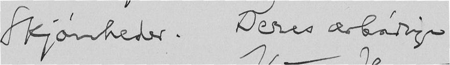Skjønheder. Deres ærbødige
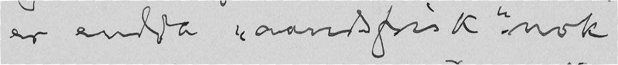er endda "aandsfrisk" nok
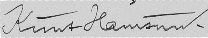Knut Hamsun.
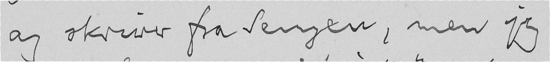og skriver fra Sengen, men jeg
